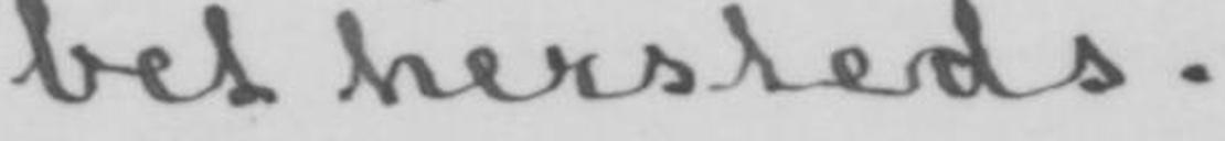bet hersteds.
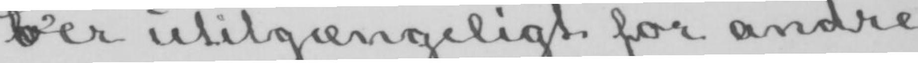bver utilgængeligt for andre
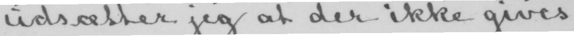udsætter jeg at der ikke gives
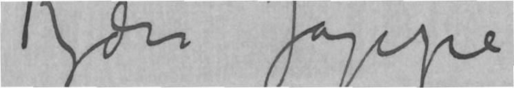Kjære Jappe
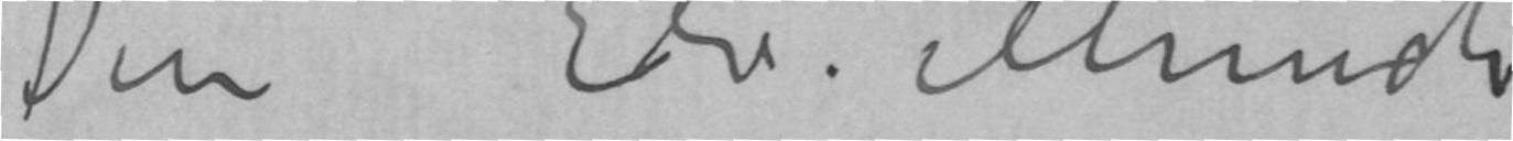Din Edv. Munch
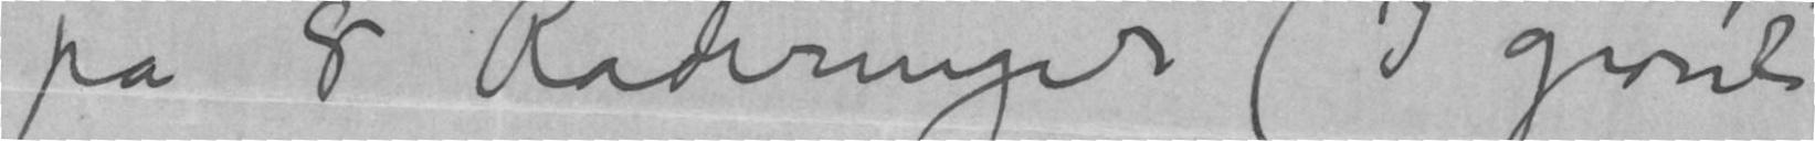på 8 Raderinger (I grønt
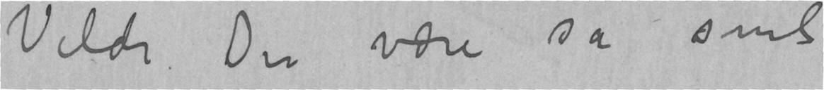Vilde Du være så snil
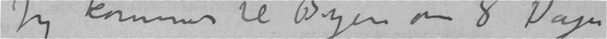Jeg kommer til Byen om 8 Dage
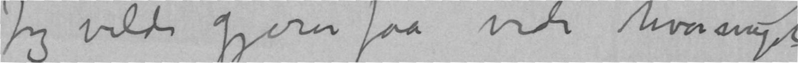Jeg vilde gjerne faa vide hvormeget
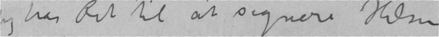jeg har Ret til at signereHilsen
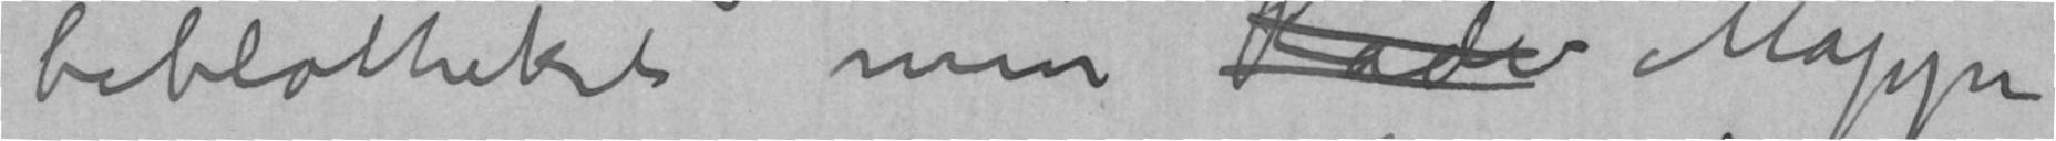biblioteket min Rader Mappe
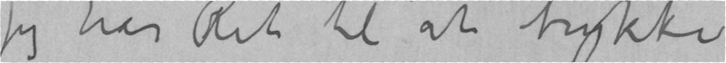jeg har Ret til at trykke
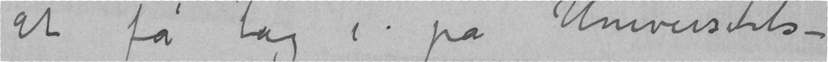at få Tag i på Universitets-
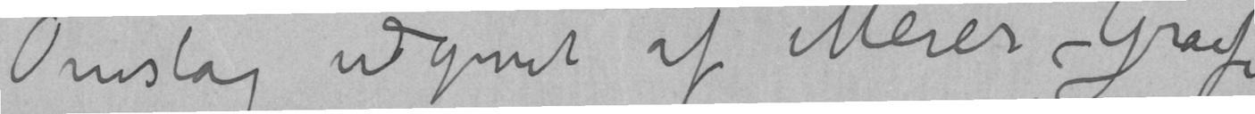Omslag udgivet af Meier-Graefe)
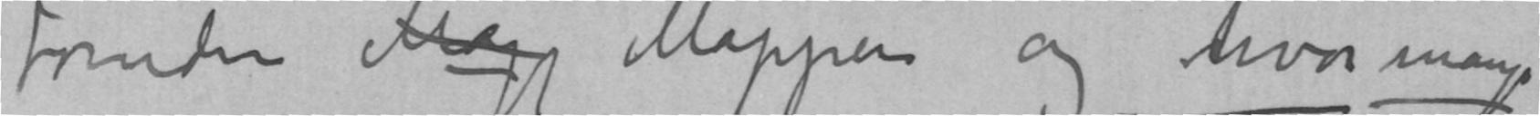foruden Map Mappen og hvor mange
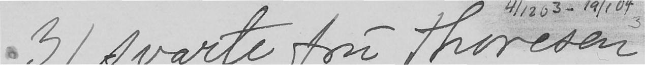3) svarte fru Thoresen
